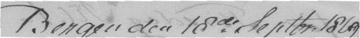Bergen den 18de Septbr. 1869
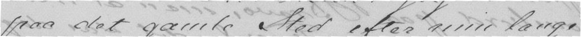paa det gamle Sted efter min lange
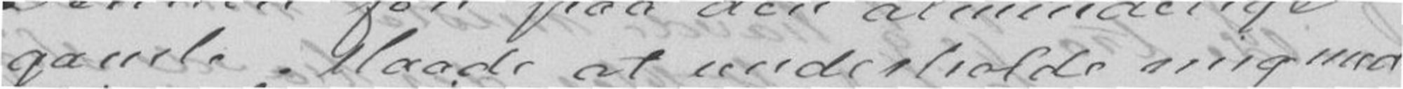gamle Maade at underholde mig med
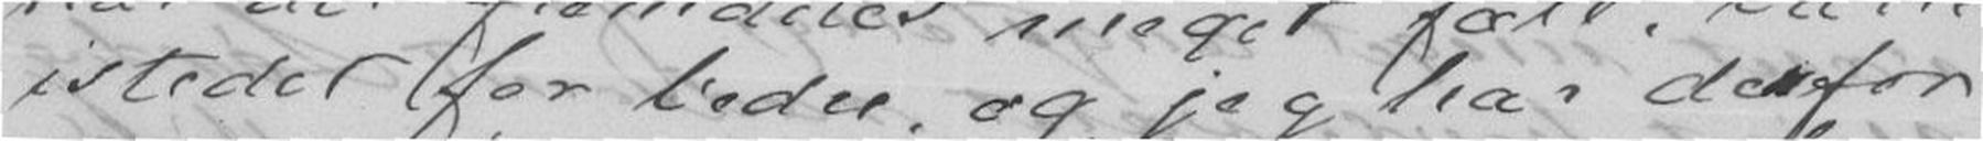istedet for beder, og jeg har derfor
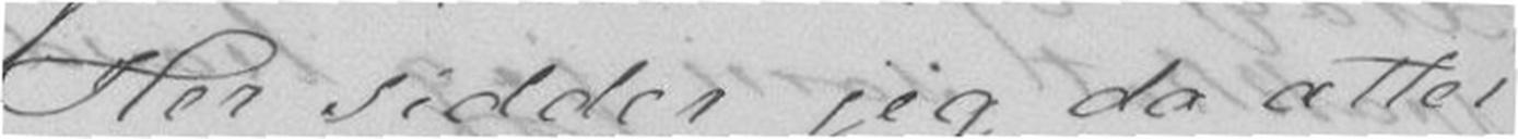Her sidder jeg da atter
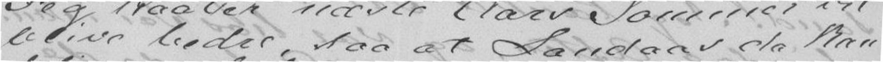blive bedre, saa at Landaas vil
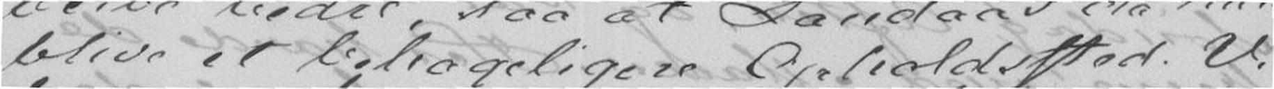blive et behageligere Opholdssted. Vi
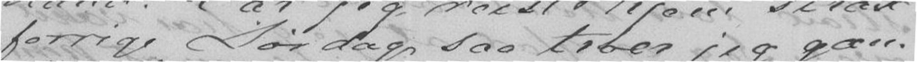forrige Lørdag saa troer jeg gan-
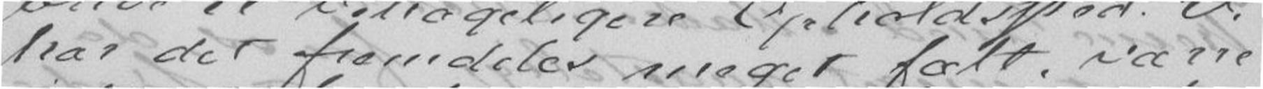har det fremdeles meget fælt, værre
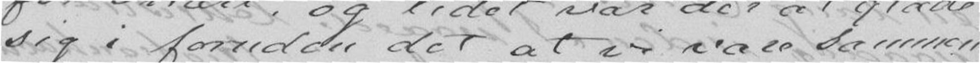sig i foruden det at vi vare sammen.
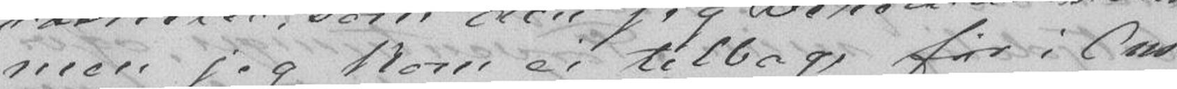men jeg kom ei tilbage før i Ons-
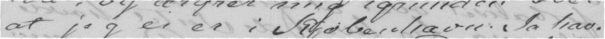at jeg ei er i Kjøbenhavn. Ja hav-
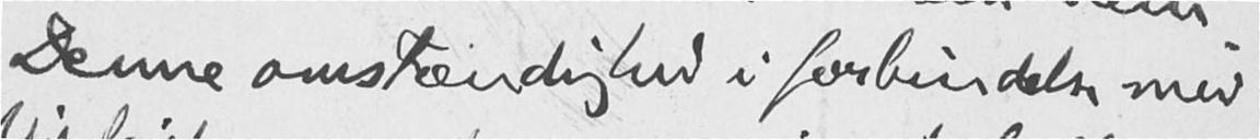Denne omstændighed i forbindelse med
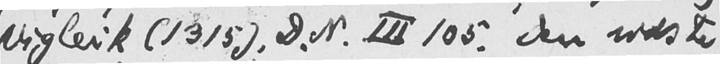Vigleik (1315); D. N. III 105. Den sidste
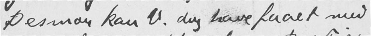Þesmon kan V. dog have faaet med
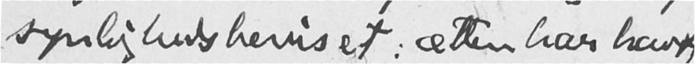synlighedsbeviset: ætten har havt
